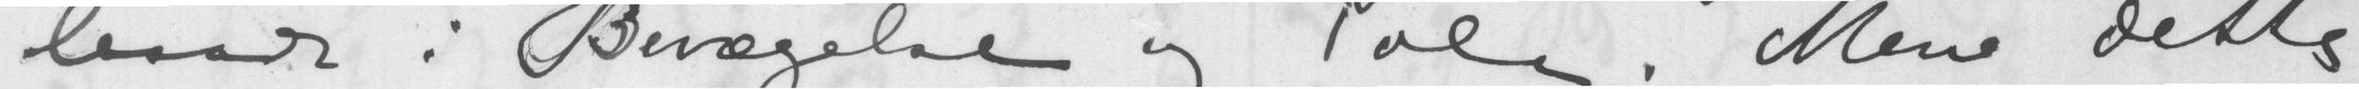baade i Bevægelse og Tale. Men dette
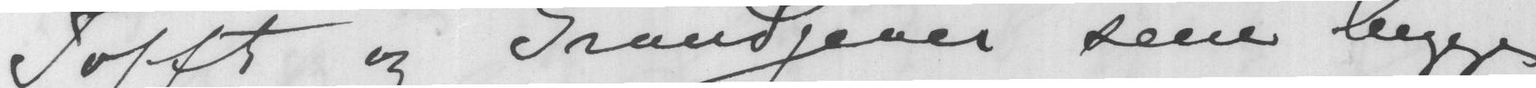Tofft og Grandjeuer som begge
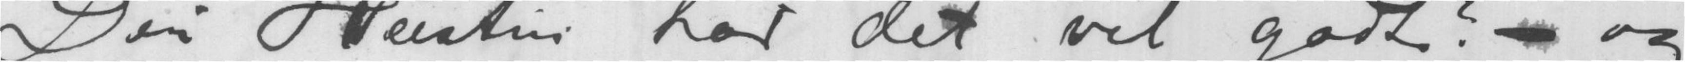Din Hustru har det vel godt? - og
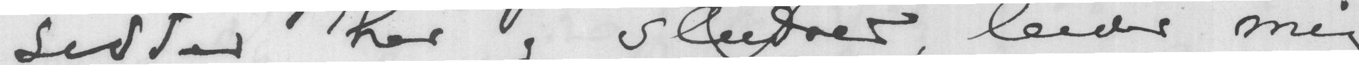sidder her og sludrer, beder mig
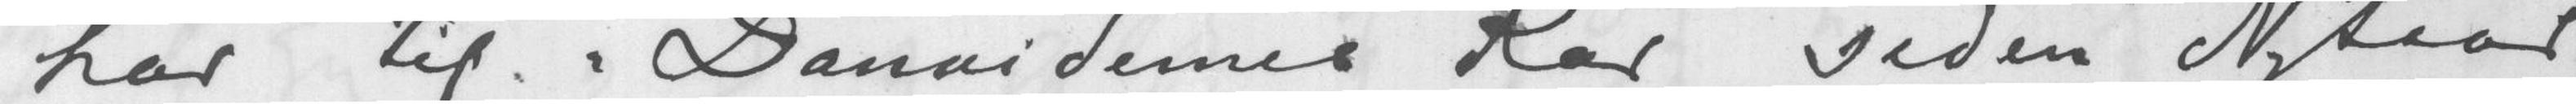har til Danaidernes Kor siden Nytaar
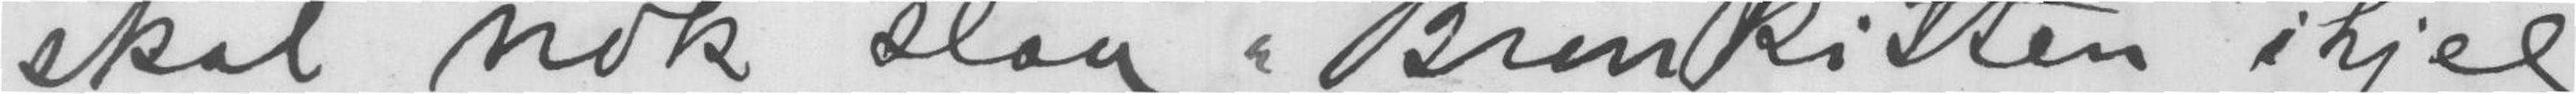skal nok slaa Bronkitten ihjel.
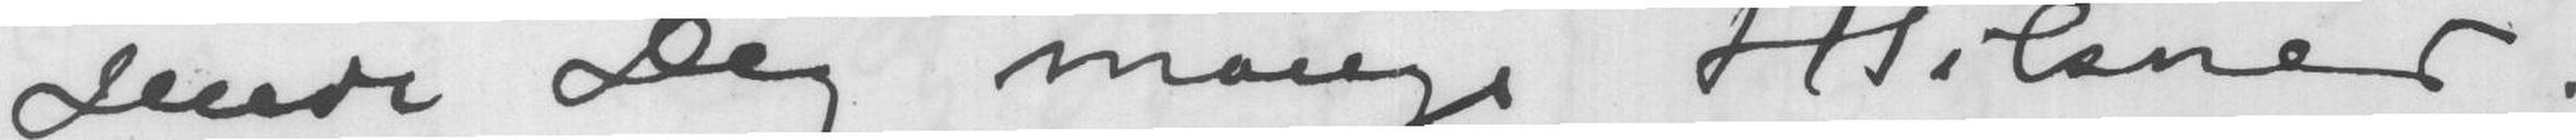sende Dig mange Hilsner.
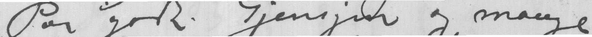Paa godt Gjensyn og mange
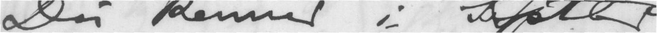Du kommer i Septbr. -
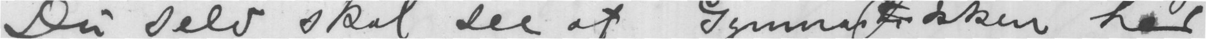Du selv skal see at Gymnastikken her
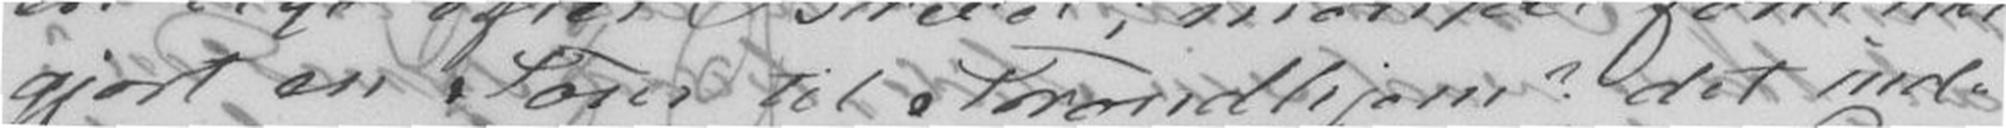gjort en Tour til Trondhjem? det ind-
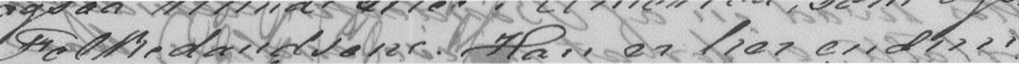Folkedandserer. Han er her endnu
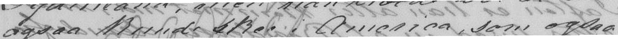ogsaa kunde skee i America, som ogsaa
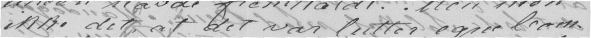ikke det at det var lutter egne Com-
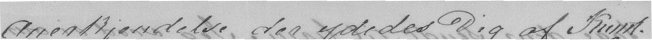Anerkjendelse der ydedes Dig af Kunst-
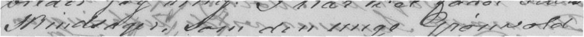Skindsager, som den unge Grønvold
